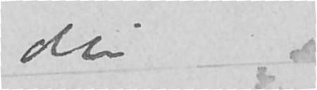din
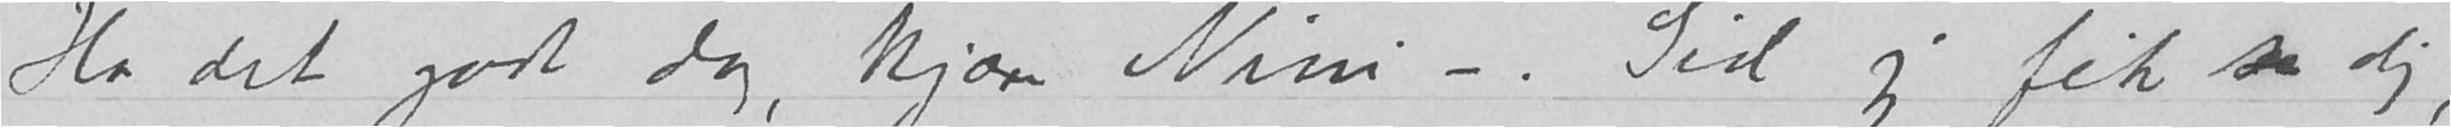Ha det godt da/dog, kjære Nini –.  Gid jeg fik se dig,
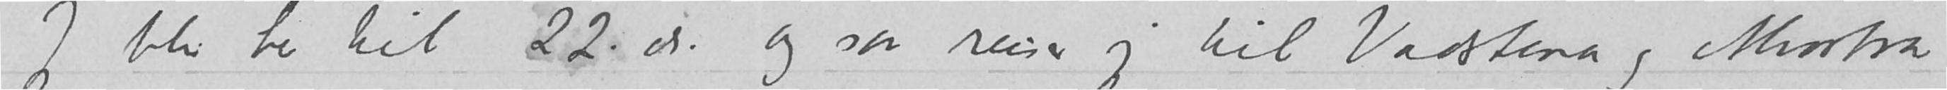Jeg blir her til 22. ds. og saa reiser jeg til Vadstena og M….
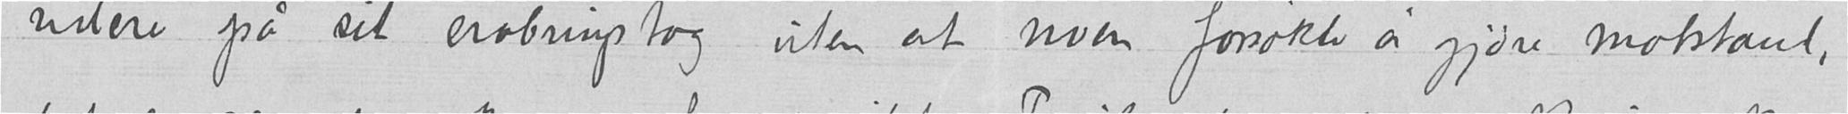videre på sit erobringstog uten at noen forsøkte å gjøre motstand,
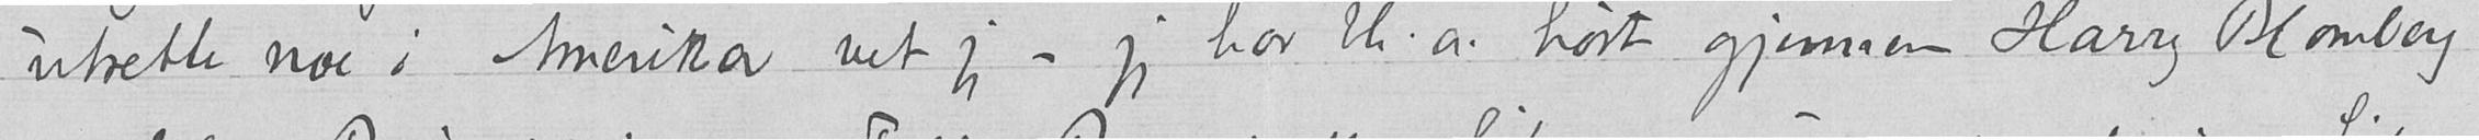utrette noe i Amerika vet jeg – jeg har bl.a. hørt gjennem Harry Blomberg
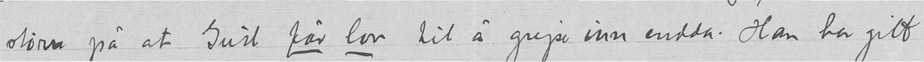større på at Gud får lov til å gripe inn endda. Han har gitt
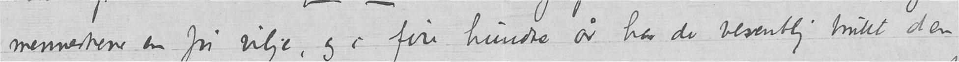menneskene en fri vilje, og i fire hundre av har de vesentlig brukt den
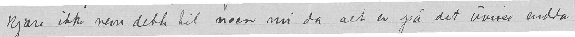kjære ikke nevn dette til noen nu da alt er på det uvisse endda
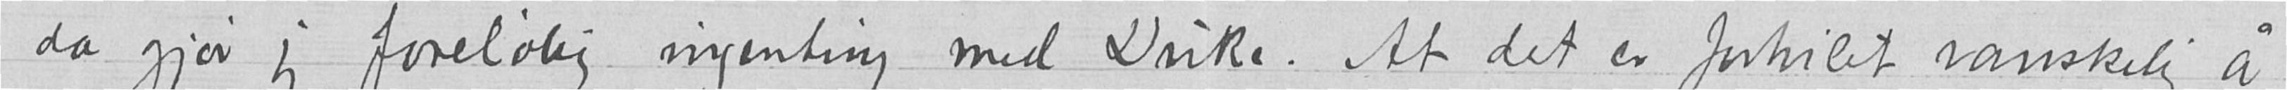da gjør jeg foreløbig ingenting med Duke. At det er fortvilet vanskelig å
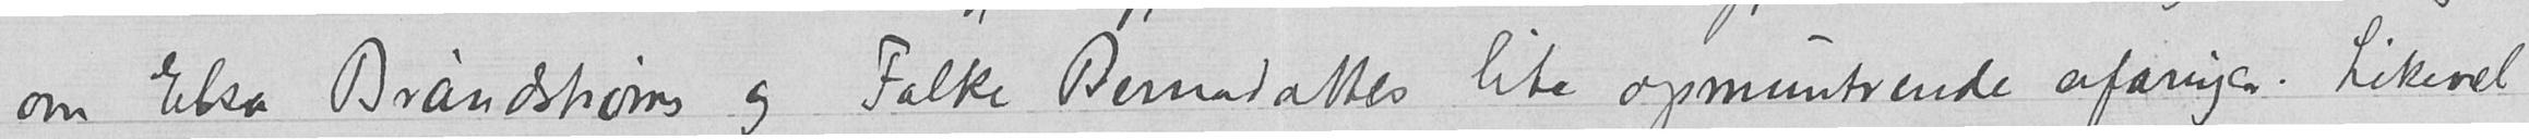om Elsa Brandstrøms og Folke Bernadottes lite opmuntrende erfaringer. Likevel
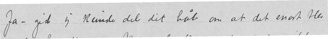Ja – gid jeg kunde dele dit håb om at det snart blev
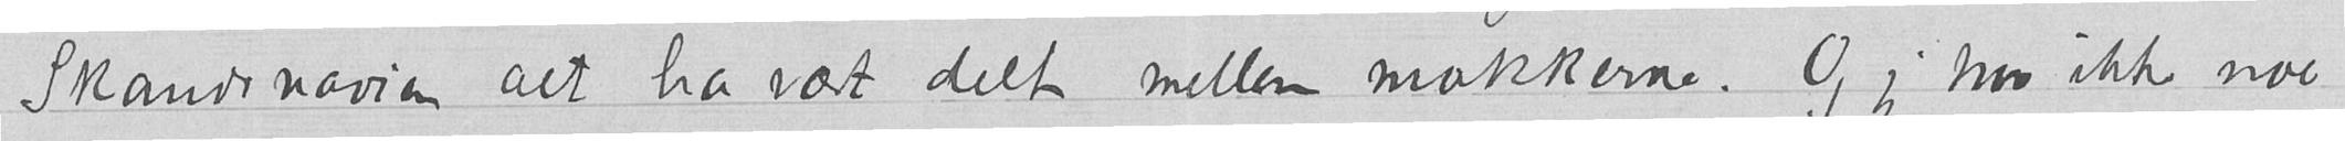Skandsnavien alt ha vært delt mellem makkerne. Og jeg tror ikke noe
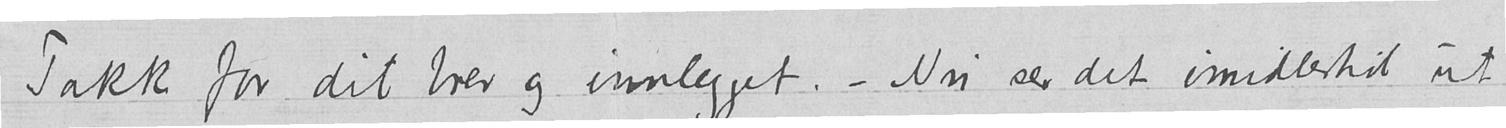Takk for dit brev og innlegget. – Nu ser det imidlertid ut
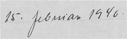15. februar 1940.
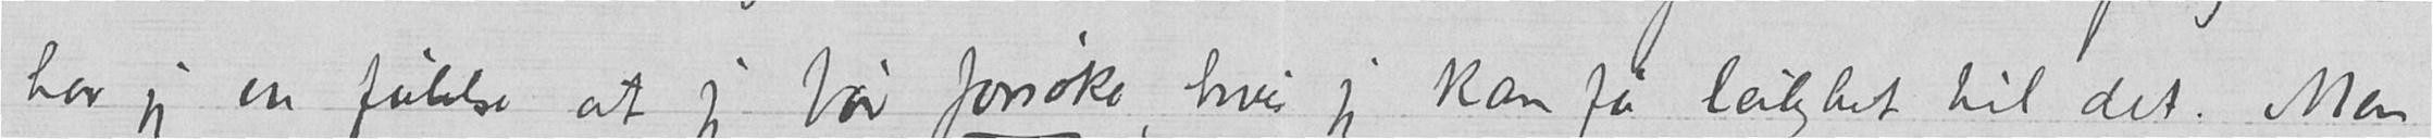har jeg en følelse at jeg bør forsøke, hvis jeg kan få leilighet til det. Men
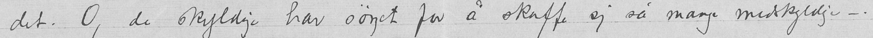det. Og de skyldige har sørget for å skaffe sig så mange medskyldige –.
